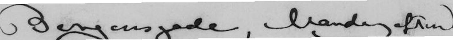Bergensgade, Mandag aften
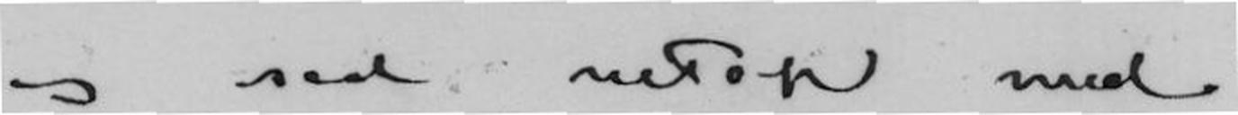Jeg sad netop med
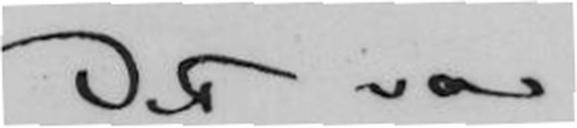Det var
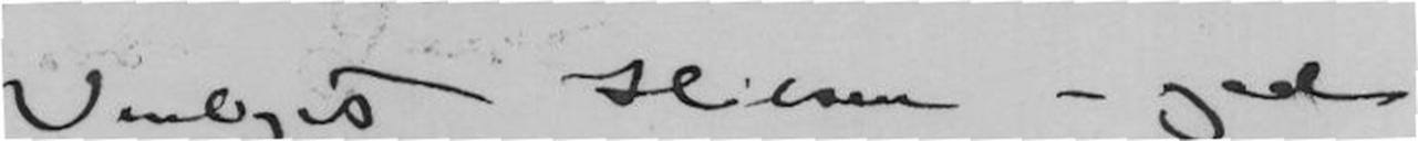Venligst Hilsen - god
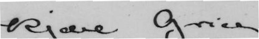Kjære Grieg
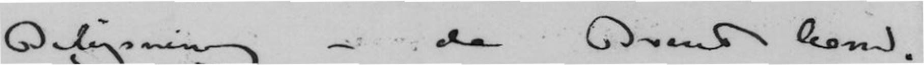Belysning - da Brevet kom.
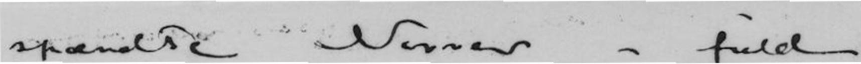spændte Nerver - fuld
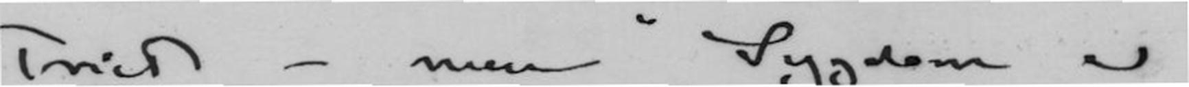trist - men "Sygdom er
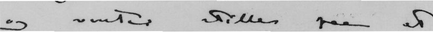og venter stille paa at
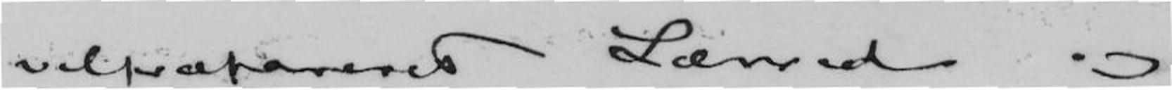velpræpareret Lærred og
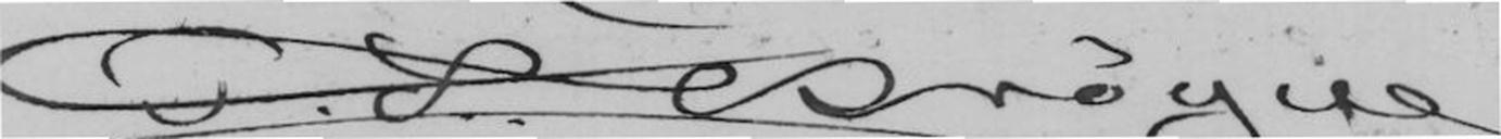P. S. Krøyer
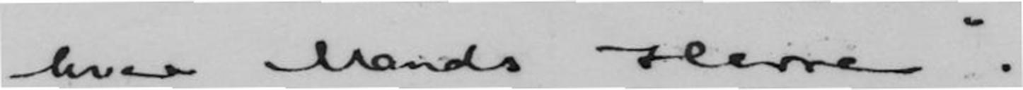hver Mands Herre".
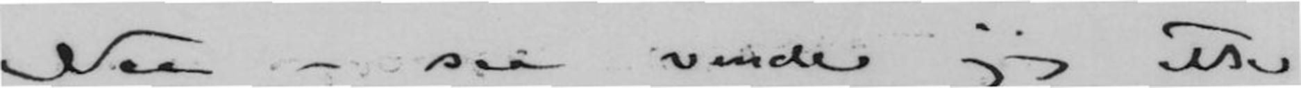Naa - saa vender jeg atter
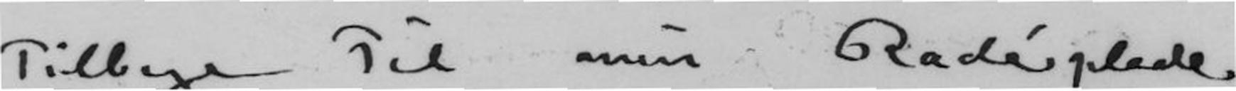tilbage til min Radérplade
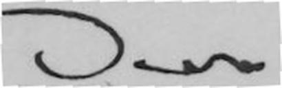Din
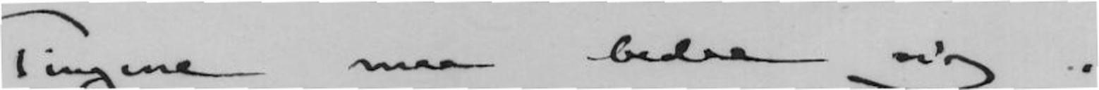Tingene maa bedre sig.
